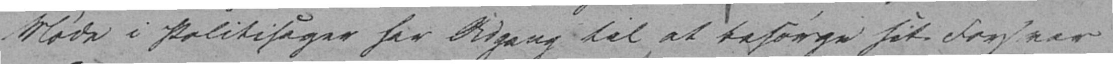Møde i Politisager har Adgang til at besørge sit Forsvar
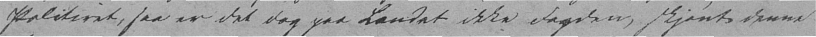Politiret, saa er det dog paa Landet ikke Fogden, skjønt denne
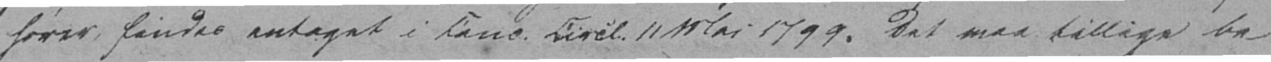hører, findes antaget i Canc. Circl. 11 Mai 1799. Det maa tillige be-
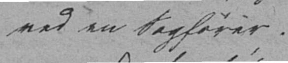ved en Sagfører.
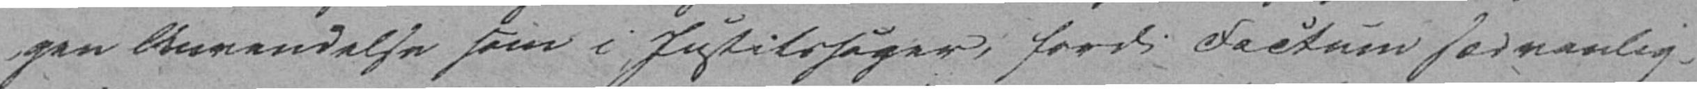gen Anvendelse som i Justitssager, fordi Factum sædvanlig-
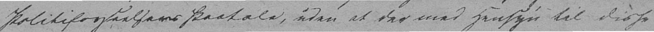Politiforseelsens Paatale, uden at der med Hensyn til disse
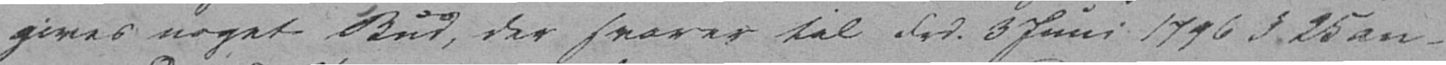gives noget Bud, der svarer til Frd. 3 Juni 1796 § 25 an-
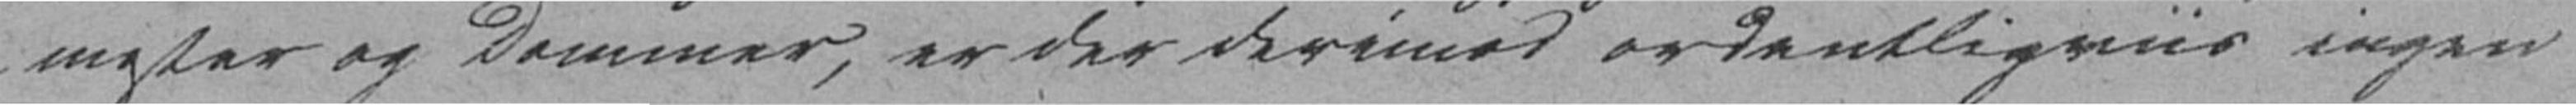mester og Dommer, er der derimod ordentligviis ingen
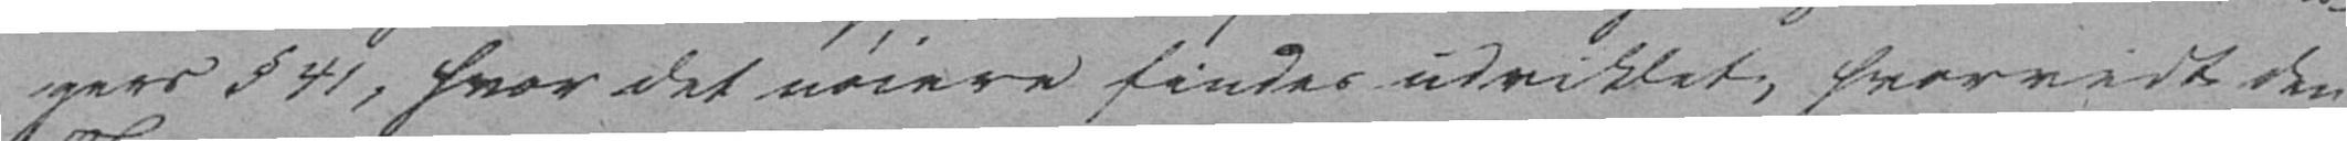gers § 41, hvor det nøiere findes udviklet, hvorved den
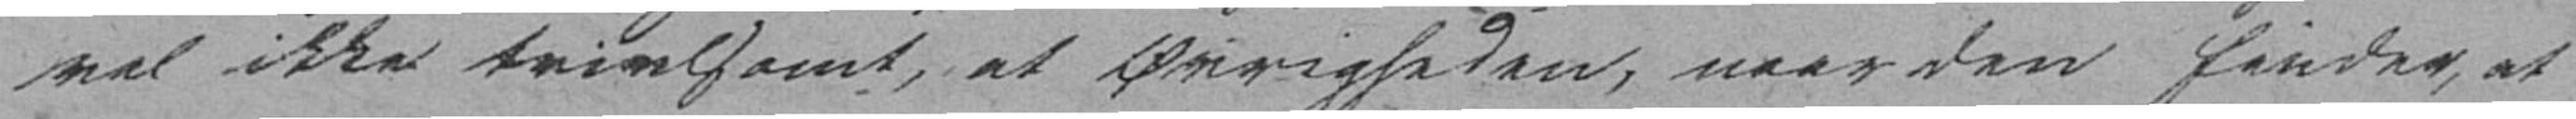vel ikke tvivlsomt, at Øvrigheden, naar den finder, at
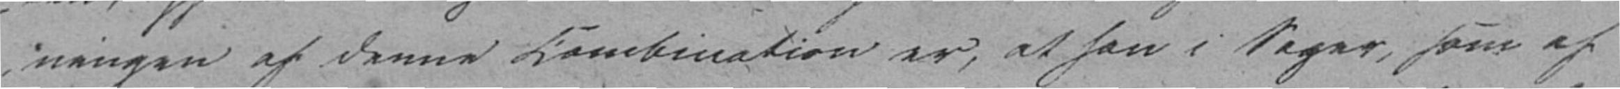ningen af denne Combination er, at han i Sager, som af
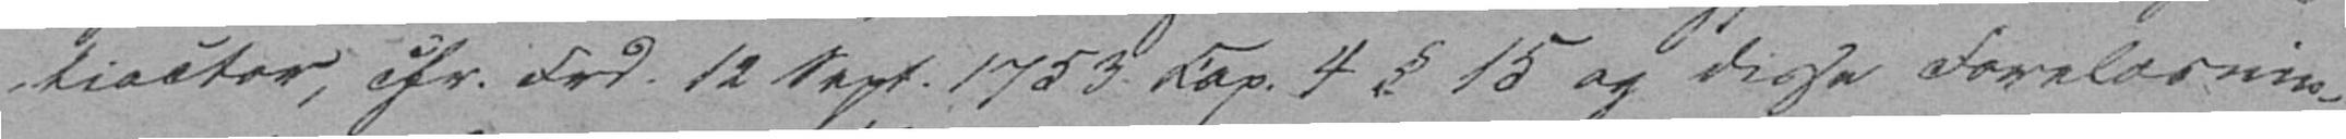tiactor, cfr. Frd. 12 Sept. 1753 Cap 4 § 15 og disse Forelæsnin-
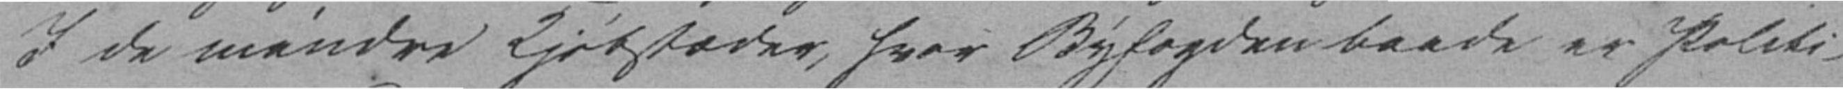I de mindre Kjøbstæder, hvor Byfogden baade er Politi-
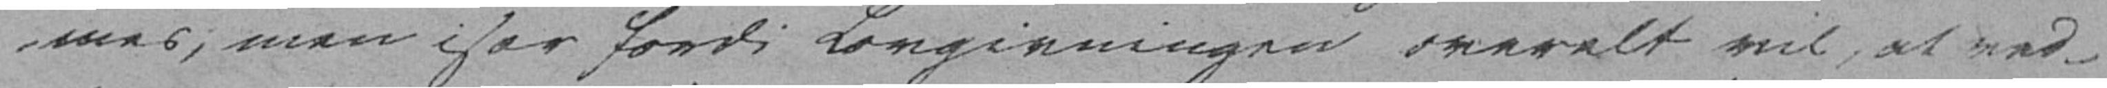mes, men især fordi Lovgivningen overalt vil, at ved-
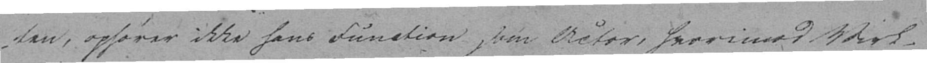ten, ophører ikke hans Function som Actor, hvorimod Virk-
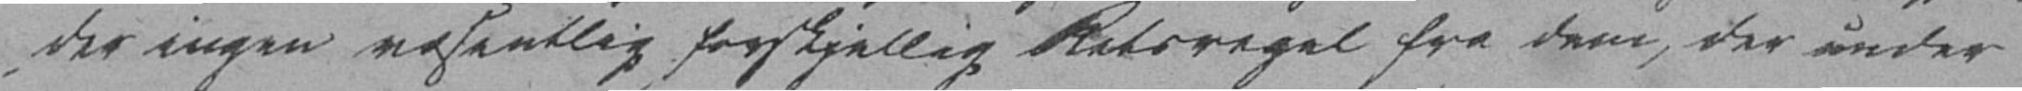der ingen væsentlig forskjellig Retsregel fra dem, der under
